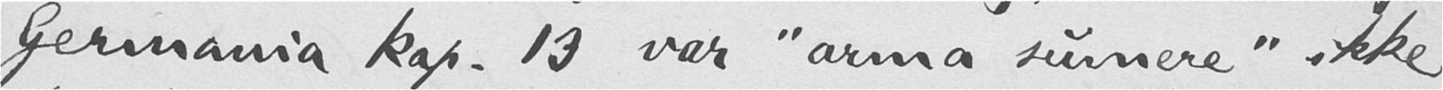Germania kap. 13 var "arma sumere" ikke
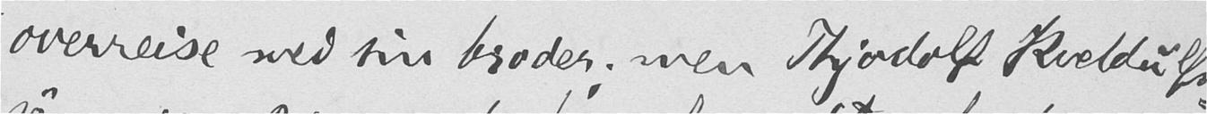overreise med sin broder; men Thjordolf Kveldulfs-
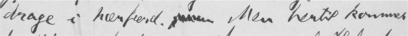drage i hærfærd.  Men hertil kommer
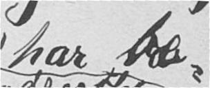har be-
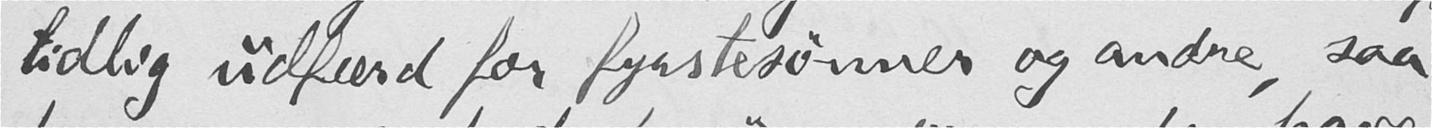tidlig udfærd for fyrstesønner og andre, saa
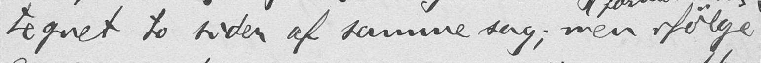tegnet to sider af samme sag; men ifølge
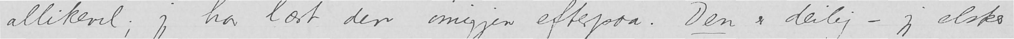allikevel; jeg har læst den omigjen efterpaa. Den er deilig – jeg elsker
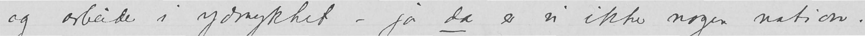og arbeide i ydmykhet –  ja da er vi ikke nogen nation.
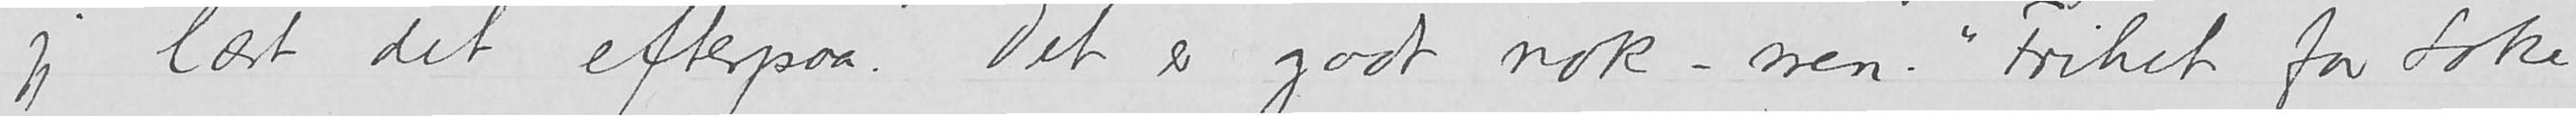jeg læst det efterpaa. Det er godt nok – men. «Frihet for
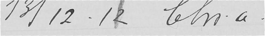13/12. 12 Chr. a.
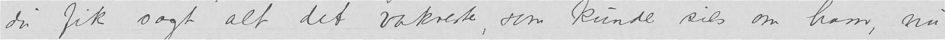du fik sagt alt det vakreste, som kunde sies om ham, nu
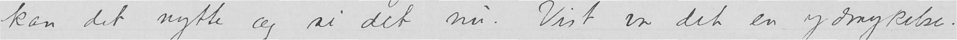kan det nytte og si det nu. Vist var det en ydmykelse.
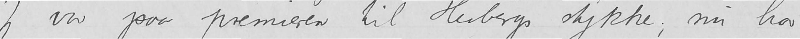Jeg var paa premieren til Heibergs stykke; nu har
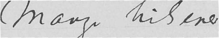Mange hilsener
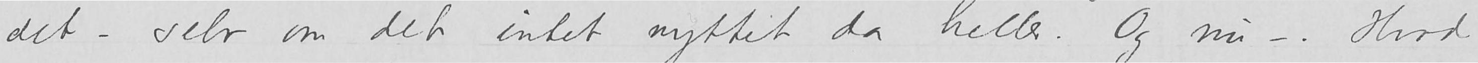det – selv om det intet nyttet da heller. Og nu –. Hvad
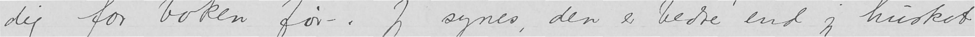dig for boken før –. Jeg synes, den er bedre end jeg husket
